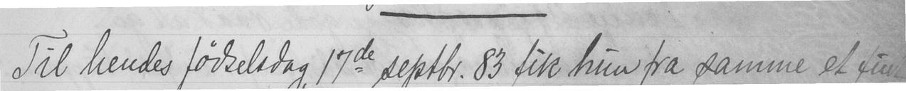Til hendes fødselsdag, 17de septbr. 83 fik hun fra samme et fint
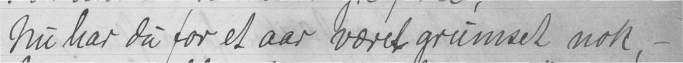nu har du for et aar været grumset nok, -
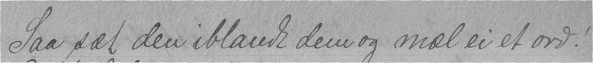Saa sæt den iblandt dem og mæl ei et ord!
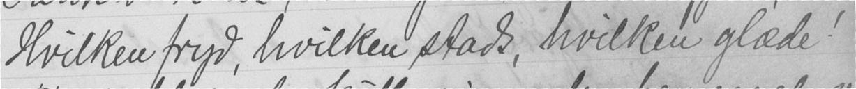Hvilken fryd, hvilken stads, hvilken glæde!
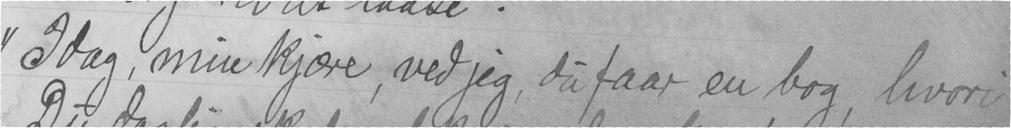"Idag, min kjære, ved jeg, du faar en bog, hvori
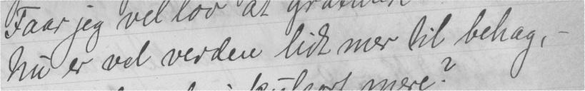Nu er vel verden lidt mer til behag,-
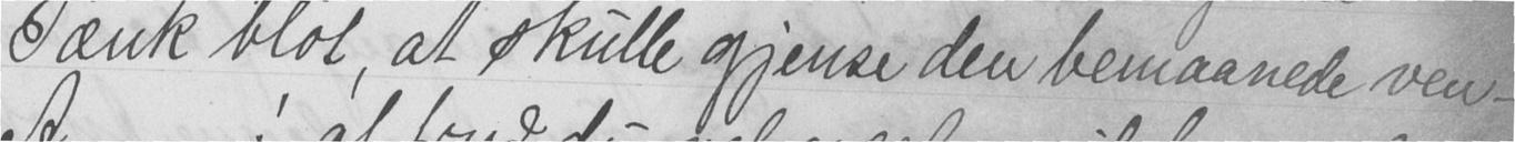Tænk blot, at skulle gjense den benaanede ven-
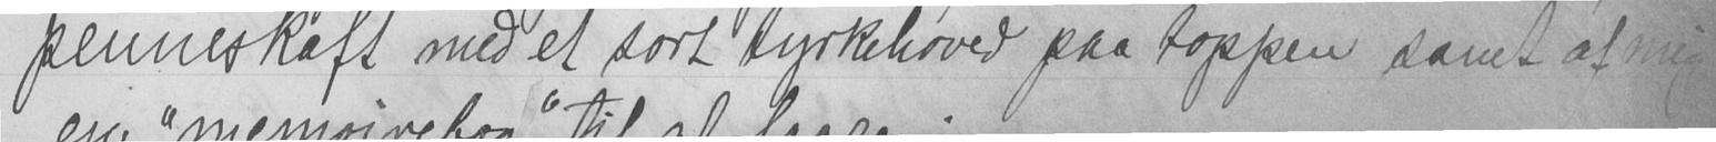penneskaft med et sort tyrkehoved paa toppen samt af mig
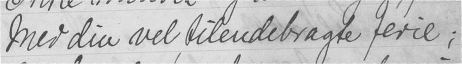Med din vel tilendebragte ferie;
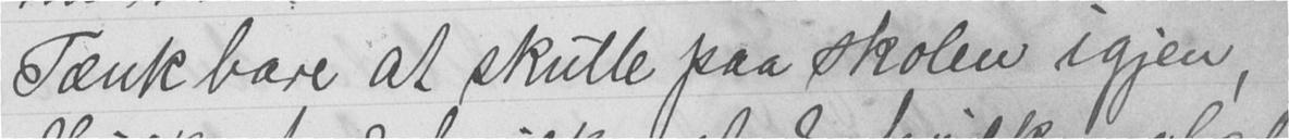Tænk bare at skulle paa skolen igjen,
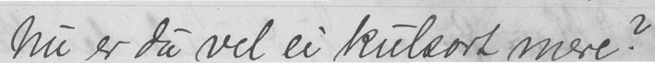nu er du vel ei kulsort mere?
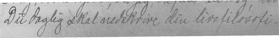Du daglig skal nedskrive din livsfilosofi.
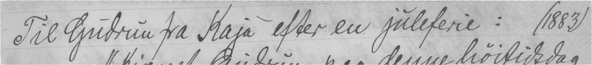Til Gudrun fra Kaja efter en juleferie: (1883)
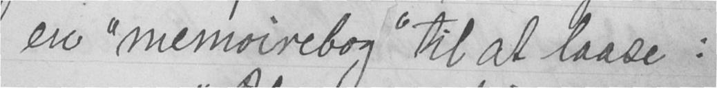en "memoirebog" til at laase :
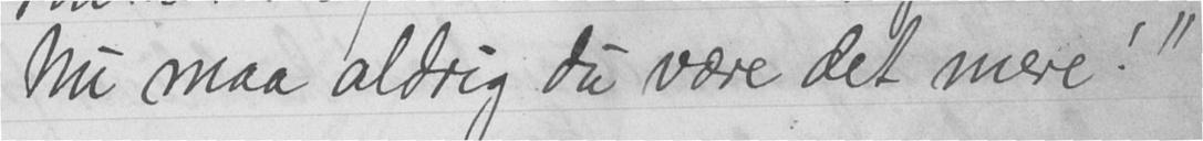nu maa aldrig du være det mere!"
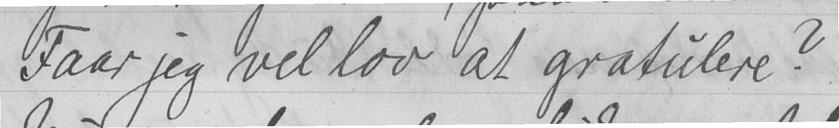Faar jeg vel lov at gratulere?
